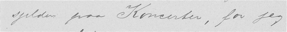sjelden paa Koncerter, for jeg
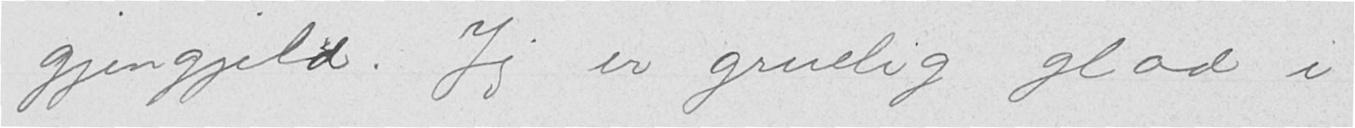gjengjeld. Jeg er gruelig glad i
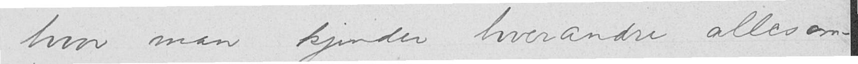hvor man kjender hverandre allesam-
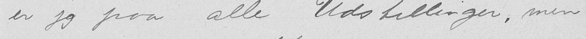er jeg paa alle Udstillinger, men
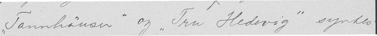"Tannhäuser" og "Fru Hedevig" syntes
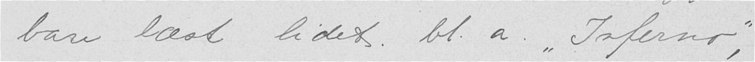bare læst lidet, bl.a. "Inferno",
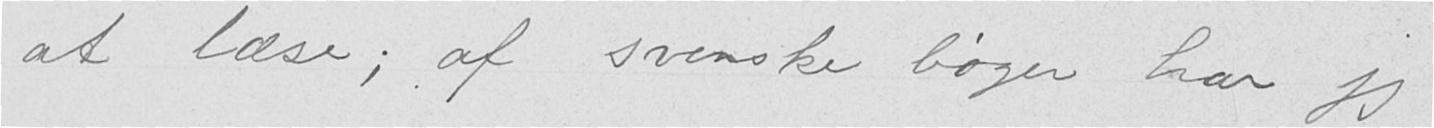i at læse; af svenske bøger har jeg
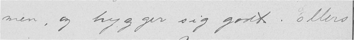men, og hygger sig godt. Ellers
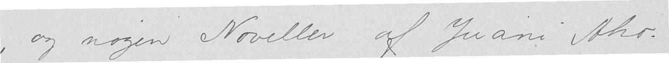og nogen Noveller af Juani Aho.
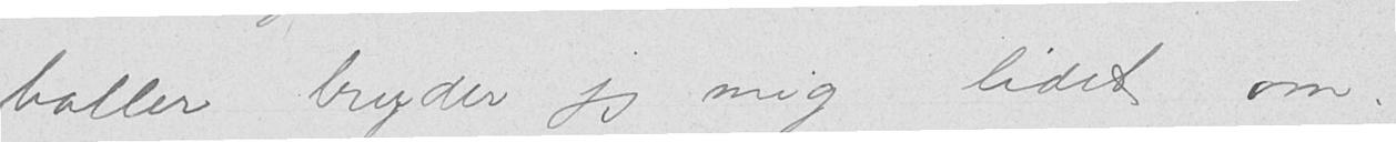baller bryder jeg mig lidet om.
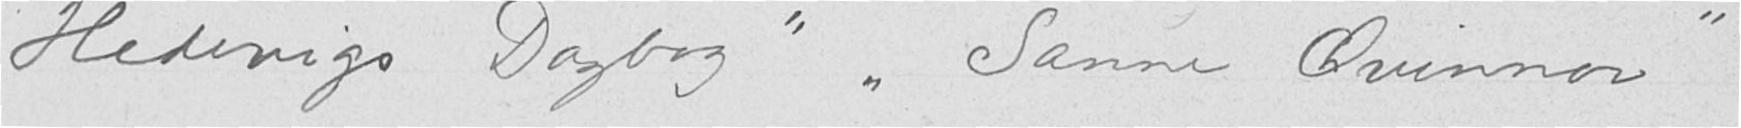Hedevigs Dagbog" "Sanne Qvinnor"
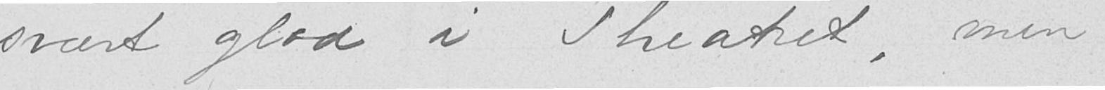svært glad i Theatret, men
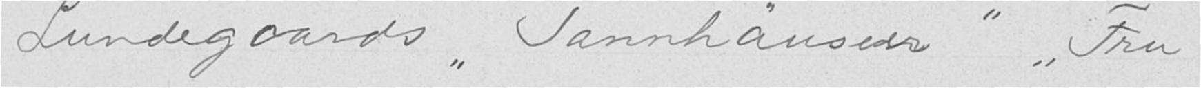Lundegaards "Tannhäuser" "Fru
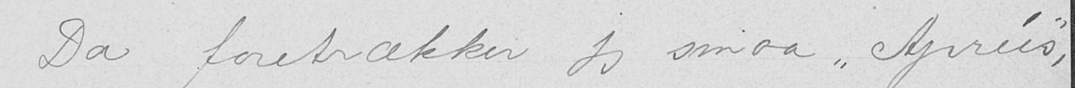Da foretrækker jeg smaa "Aprées",
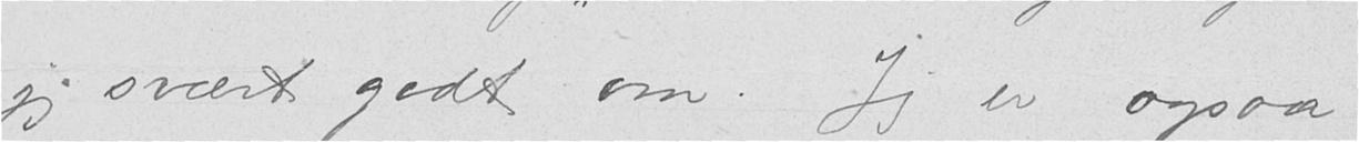jeg svært godt om. Jeg er ogsaa
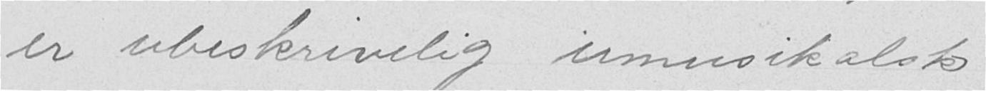er ubeskrivelig umusikalsk.
